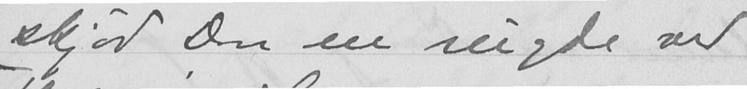skjød Don en rugde ved
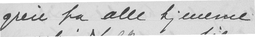greie fra alle tjenerne
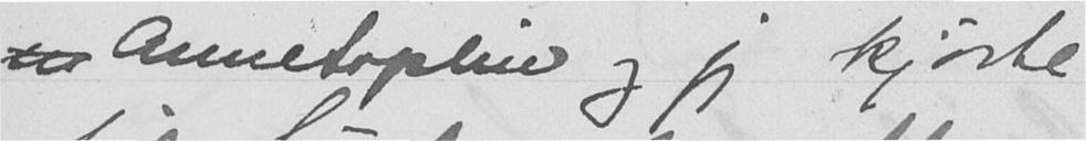an Anne Sophie og jeg kjørte
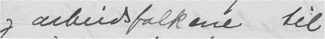og arbeidsfolkene til
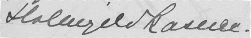Holmgildkasene.
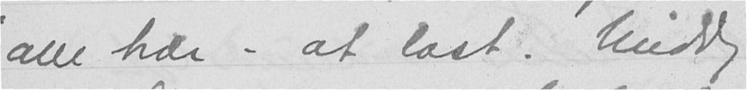alle trær - at last. Middag
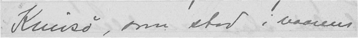Knivsø, som stod i vaarens
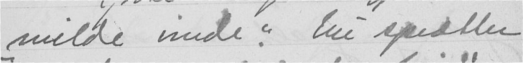"milde vinde". Nu sprætter
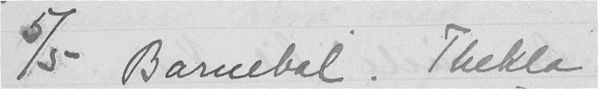5/5 Barnebal. Thekla
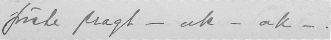første pragt - ak - ak - .
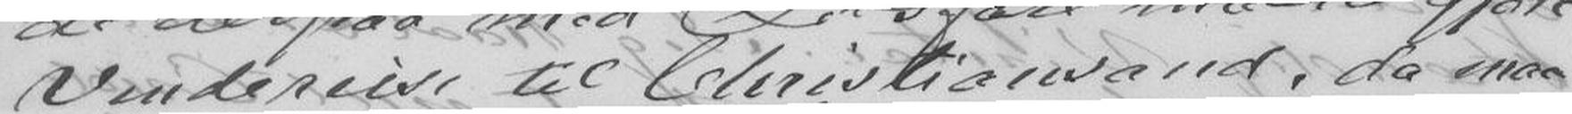Vendereise til Christiansand, da maa
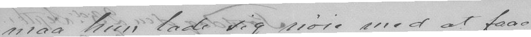maa hun lade sig nøie med at faae
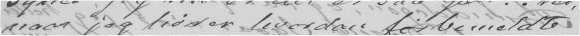naar jeg hører hvordan førbemeldte
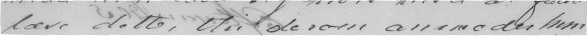læse dette, thi derom anmoder hun
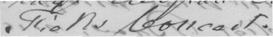Ficks Concert.
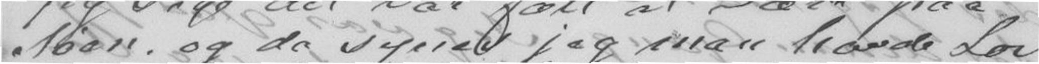Søen, og da synes jeg man havde Lov
